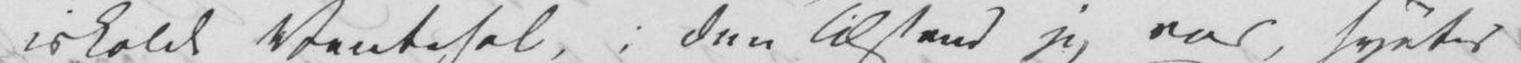iskolde Ventesal, i den Tilstand jeg var, syntes
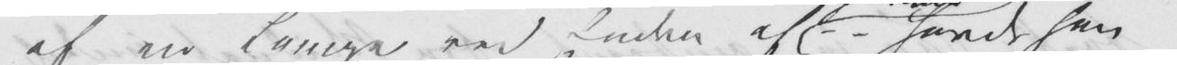af en Lampe ved Enden af – – havde han
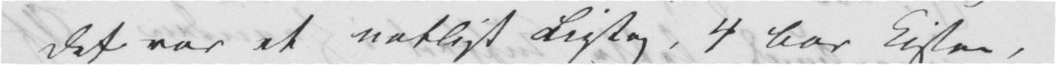Det var et natligt Ligtog, 4 bar Kisten,
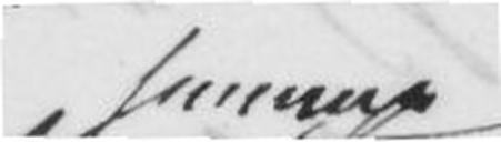samme
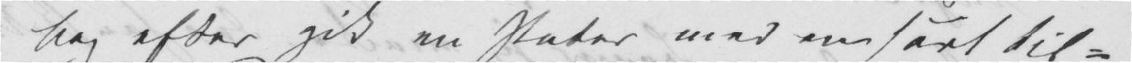bag efter gik en Pater med en sort til¬
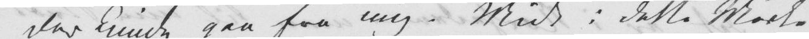der kunde gaa fra mig. Midt i dette Mørke
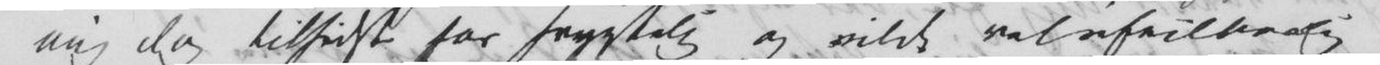mig dog tilsidst for frygtelig og vilde vel ufeilbarlig
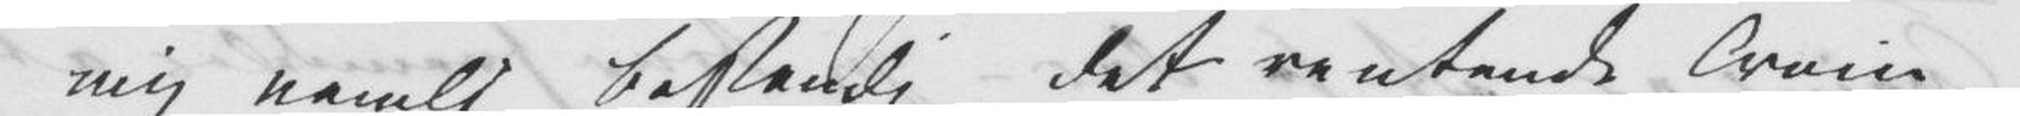mig nemlig bestandig det ventende Train
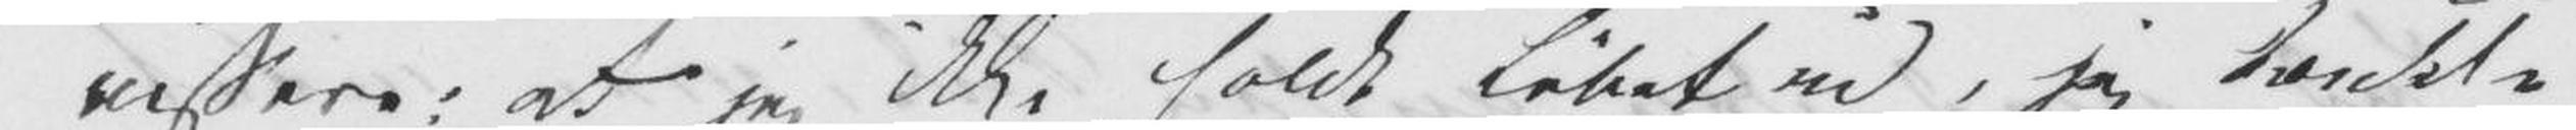vissere: at jeg ikke holdt Løbet ud, jeg tænkte
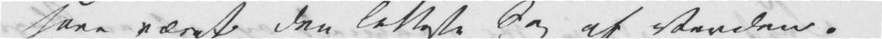have været den letteste Sag af Verden.
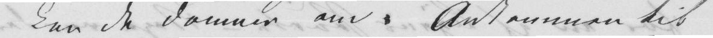kan det dømmes om. Ankommen til
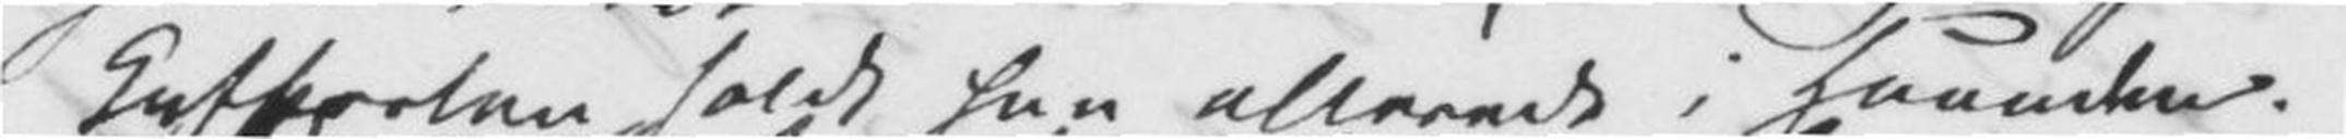Kufferten holdt han allerede i Haanden.
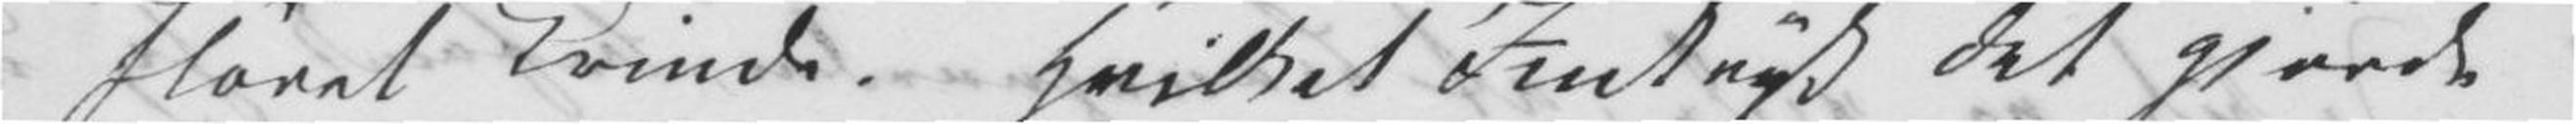sløret Kvinde. Hvilket Indtryk det gjorde
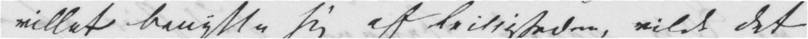villet benytte sig af Leiligheden, vilde det
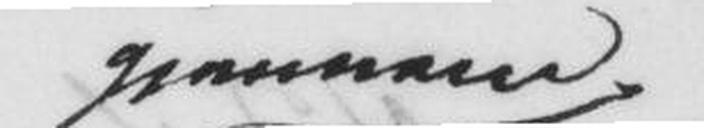gjennem
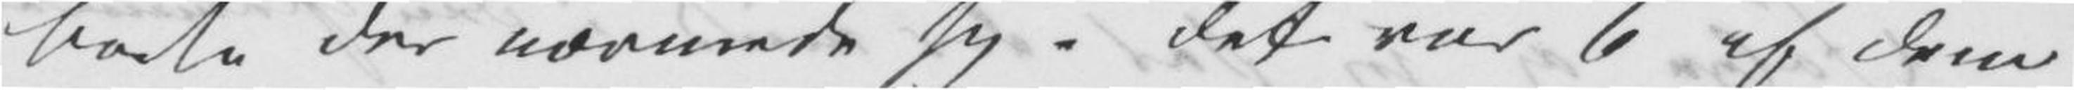borte der nærmede sig. Det var 6 af dem
